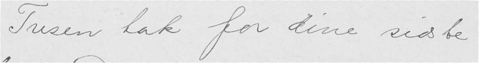Tusen tak for dine sidste
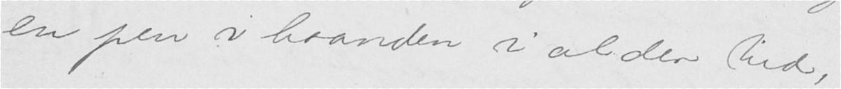en pen i haanden i al den tid,
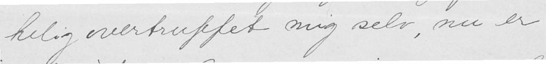kelig overtruffet mig selv, nu er
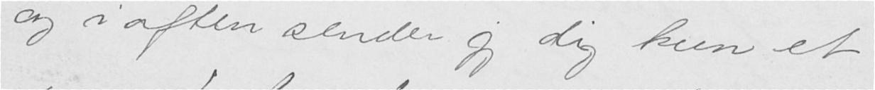og i aften sender jeg dig kun et
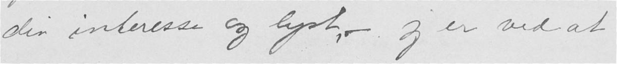din interesse og lyst, - jeg er ved at
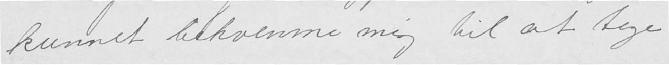kunnet bekvemme mig til at tage
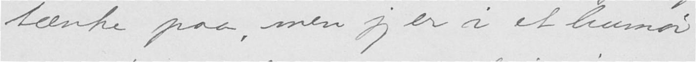tænke paa, men jeg er i et humør
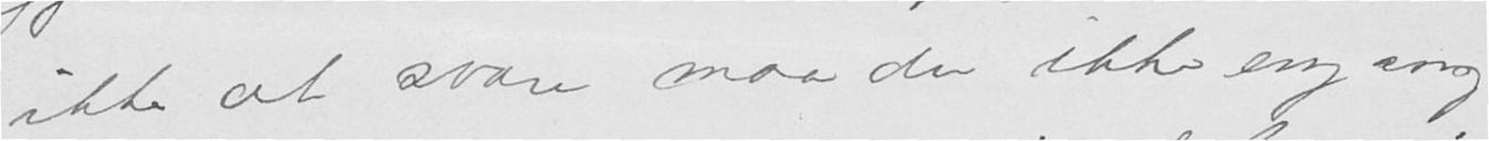ikke at svare maa du ikke engang
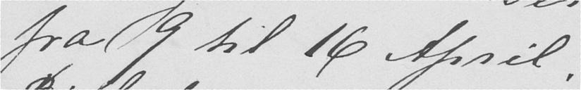fra 9 til 16 April
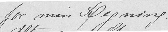for min Regning.
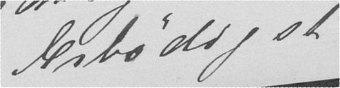Ærbødigst
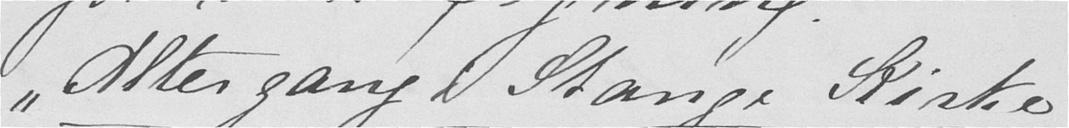"Altergang i Stange Kirke"
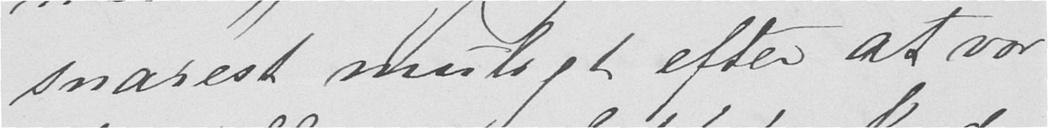snarest muligt efter at vor
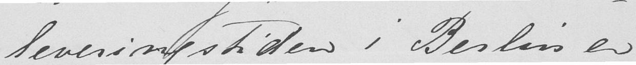leveringstiden i Berlin er
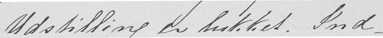Udstilling er lukket. Ind-
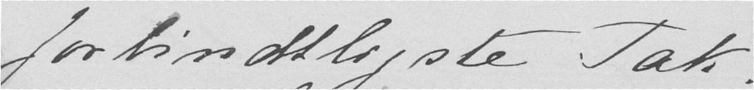forbindtligste Tak.
